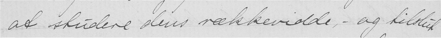at studere dens rækkevidde, - og tilslut
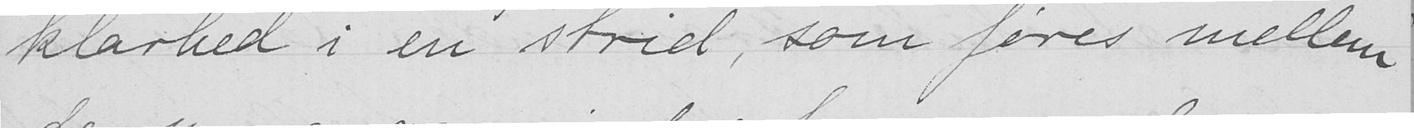klarhed i en strid, som føres mellem
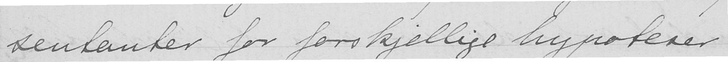sentanter for forskjellige hypoteser
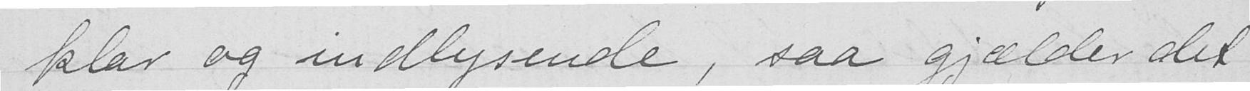klar og indlysende, saa gjælder det
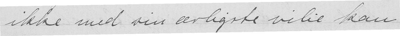ikke med sin ærligste vilie kan
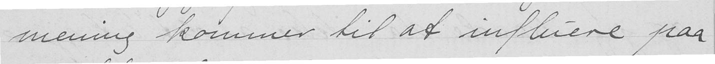mening kommer til at influere paa
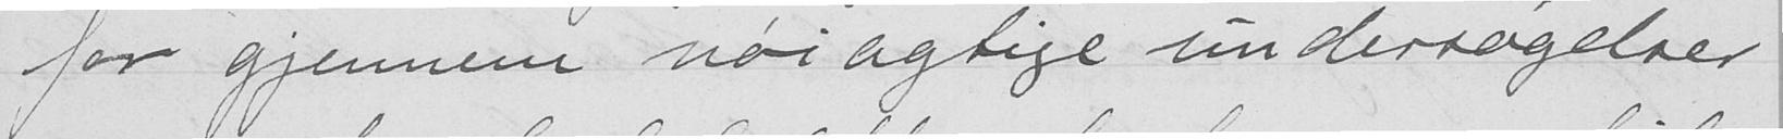for gjennem nøiagtige undersøgelser
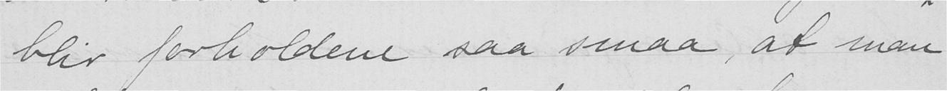blir forholdene saa smaa, at man
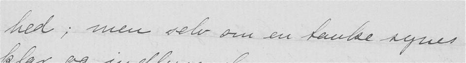hed; men selv om en tanke synes
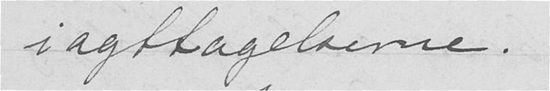iagttagelserne.
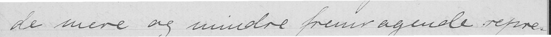de mere og mindre fremragende repre-
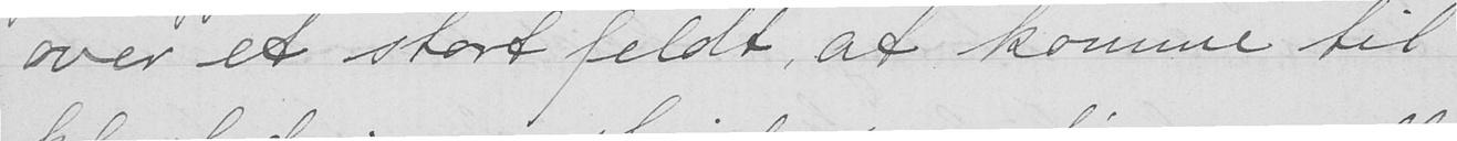over et stort feldt, at komme til
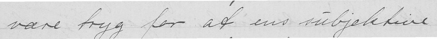være tryg for at ens subjektive
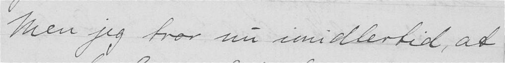Men jeg tror nu imidlertid, at
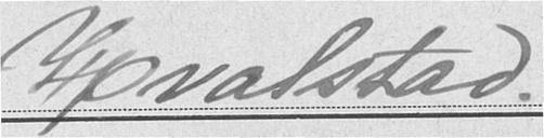Hvalstad
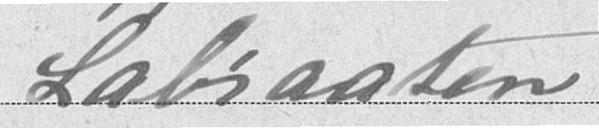Labraaten
